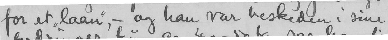for et "laan" - og han var beskeden i sine
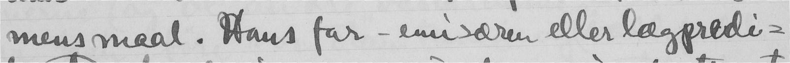mens maal. Hans far - emisæren eller lægpredi-
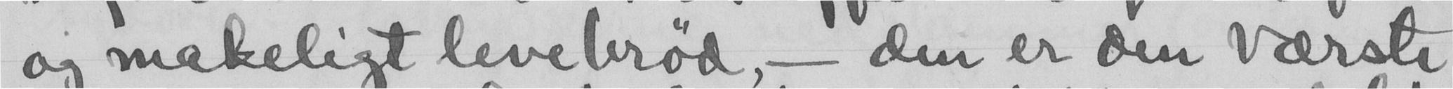og makeligt levebrød, - den er den værste
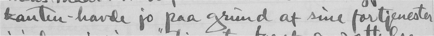kanten - havde jo paa grund af sine fortjenester
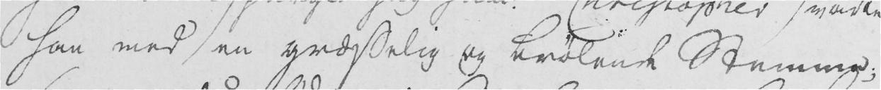han med en græsselig og brølende Stemme;
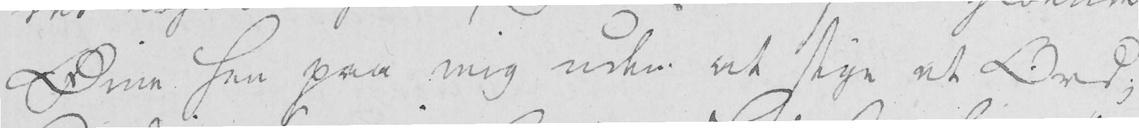Øine hen paa mig uden at sige et Ord;
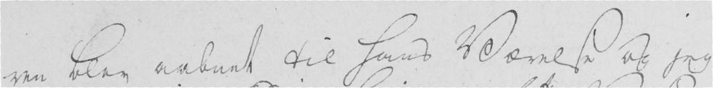ren blev aabnet til hans Værelse og jeg
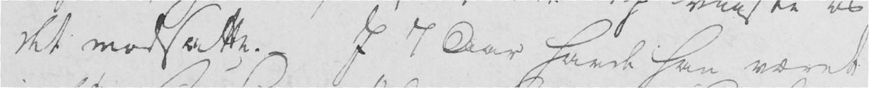det modsatte. I 7 Aar havde han været
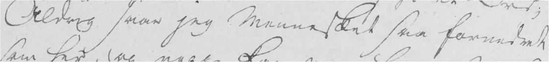Aldrig saae jeg Mennesket saa fornedret
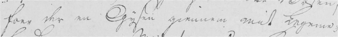foer der en Gysen giennem mit Legeme;
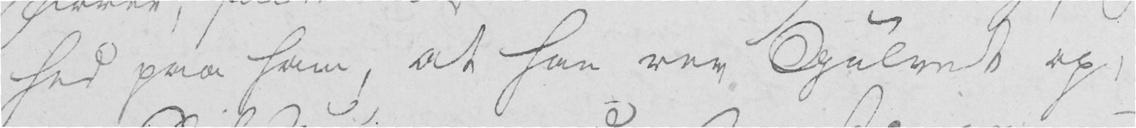hed paa ham, at han rev Gulvet op,
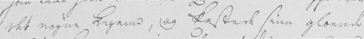det nøgne Legeme, og kastede sine gloende
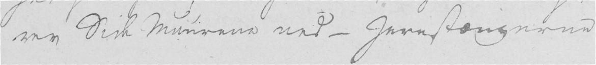rev Side-Muurene ned - Jernstængerne
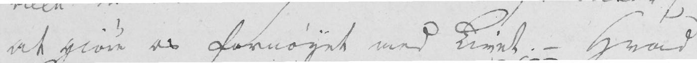at giøre os fornøyet med Livet. - Hvad
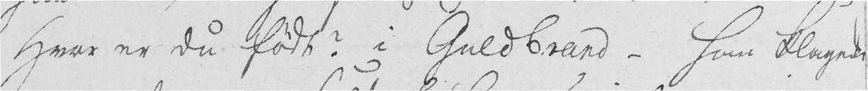Hvor er du født? i Guldbrand - Han klagede
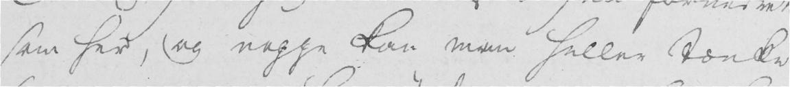som her, og neppe kan man heller tænke
